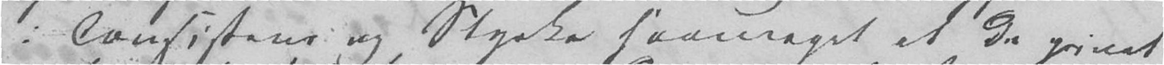i Consistens og Styrke saameget at de privat-
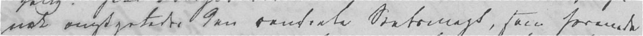nok omstyrtedes den ndente Statsmagt, som forende
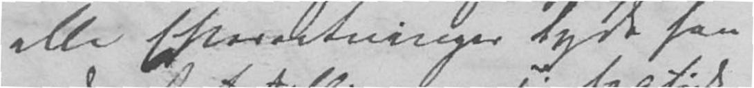alle Efterretninger tyde hen
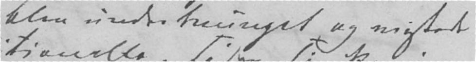blev undertvunget og mistede
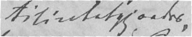tilintetgjordes,
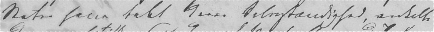Stater have tabt deres Selvændighed, enkelte
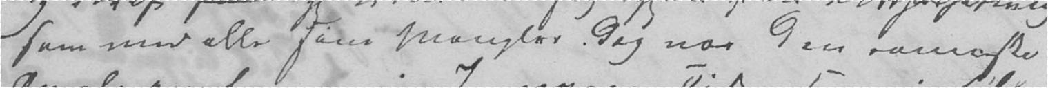som med alle sine Mangler dog var den romerske
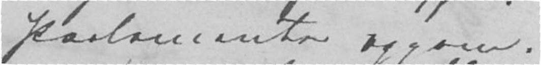Parlamenter opgave i
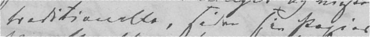traditionelle, siden sin Papier
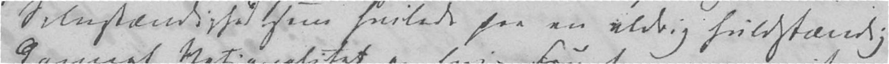Selvstændighed som hvilede paa en aldrig fuldstændig
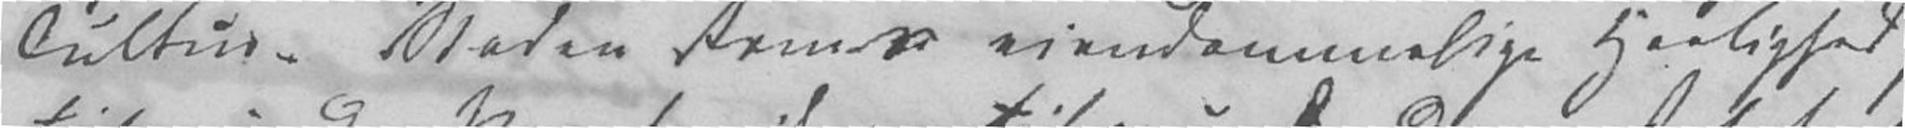Cultur. Staden Roms eiendommelige Herlighed
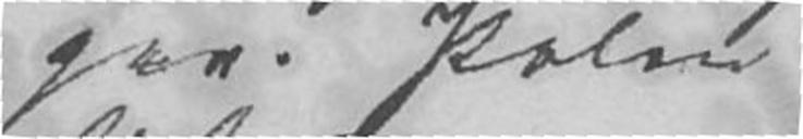ger. Polen
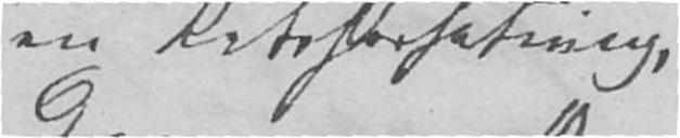en Retsforfatning,
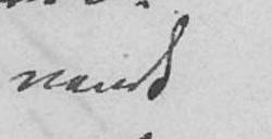vand
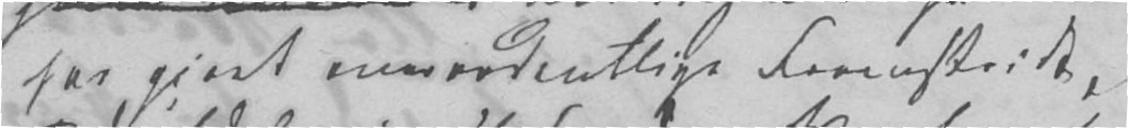har gjort overordentlige Fremskridt,
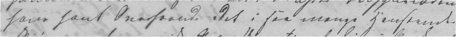haver havt Overhaand. Det i saa mange Henseende
